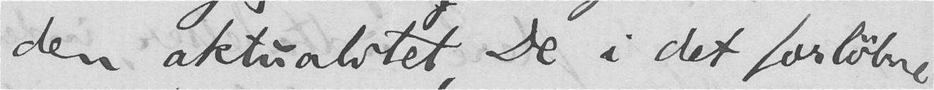den aktualitet, De i det forløbne
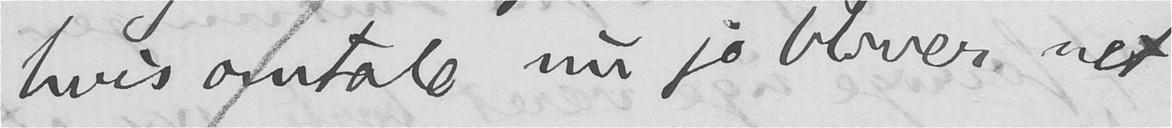hvis omtale nu jo bliver net
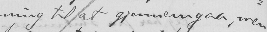ning til at gjennemgaa, men
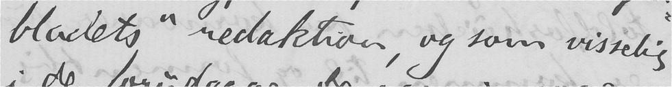bladets" redaktion, og som visselig
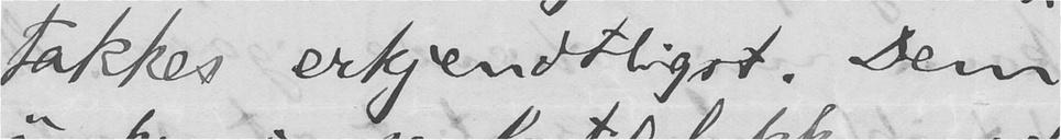takkes erkjendtligst. Dem
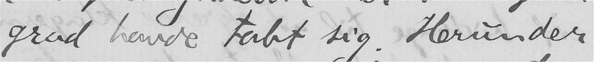grad havde tabt sig. Herunder
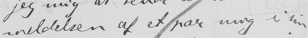meldelsen af et par mig i sin
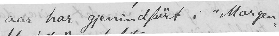aar har gjenindført i "Morgen-
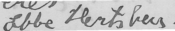Ebbe Hertzberg.
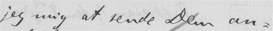jeg mig at sende Dem an-
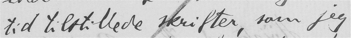tid tilstillede skrifter, som jeg
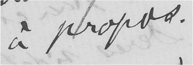à propos.
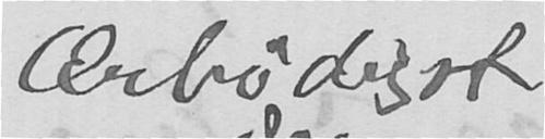Ærbødigst
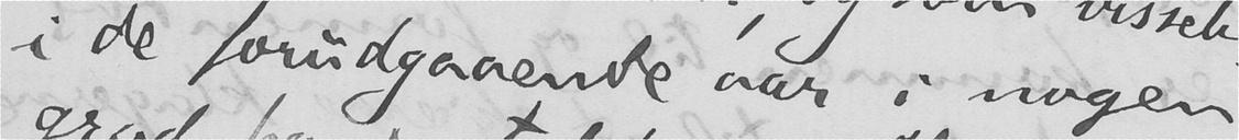i de forudgaaende aar i nogen
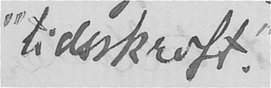"tidsskrift."
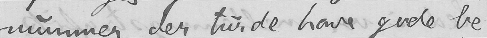nummer, der turde have gode be-
